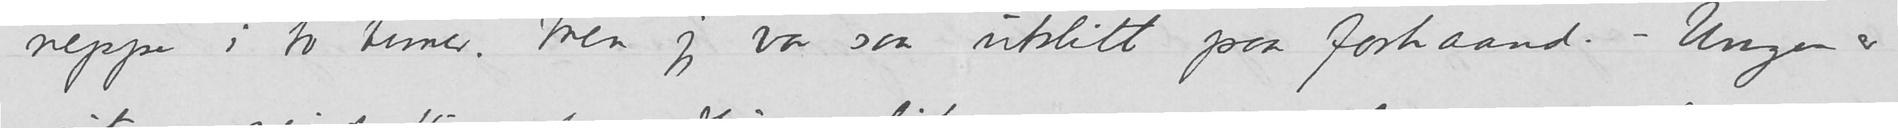neppe i to timer. Men jeg var saa utslitt paa forhaand. – Ungen er
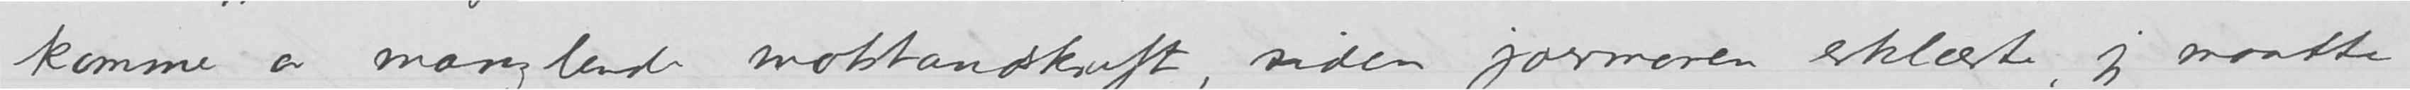komme ev manglende motstandskraft, siden jormoren erklærte, jeg maatte
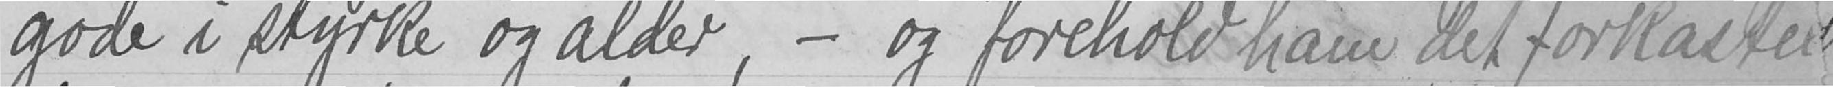gode i styrke og alder, - og forehold ham det forkaste
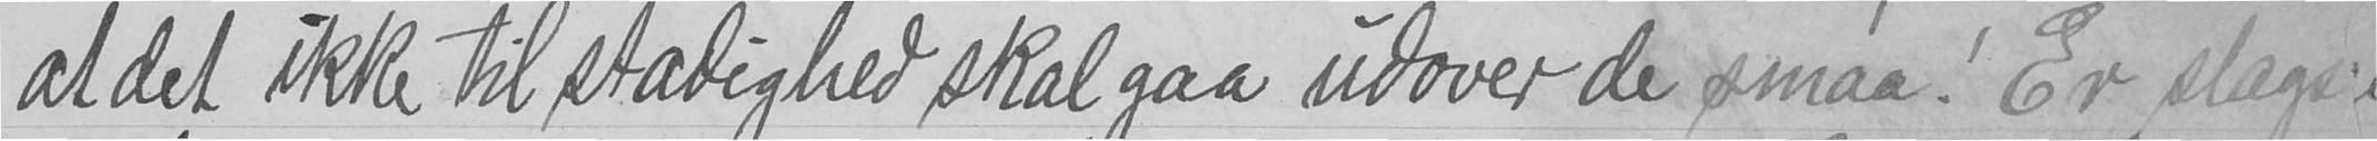at det ikke til stadighed skal gaa udover de smaa! Er slagsmål
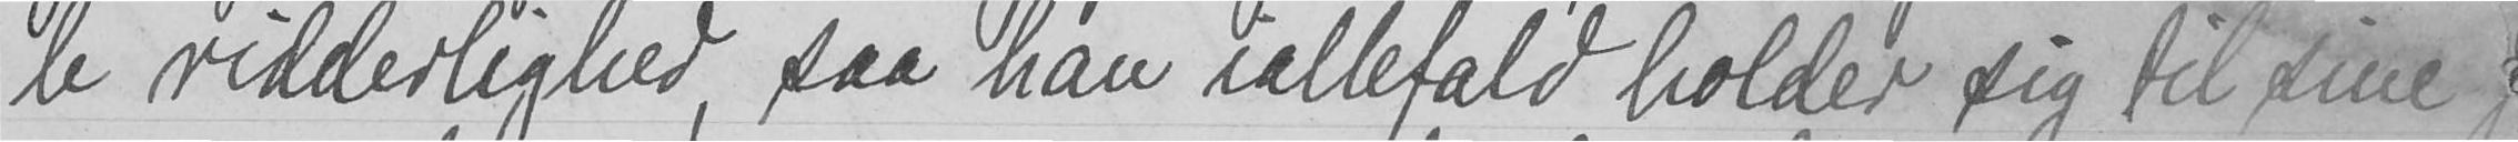le ridderilghed, saa han iallefald holder sig til sine
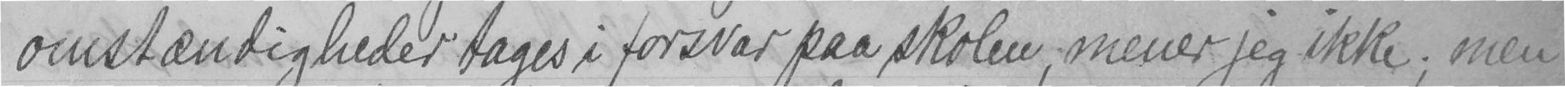omstændigheder tages i forsvar paa skolen, mener jeg ikke; men
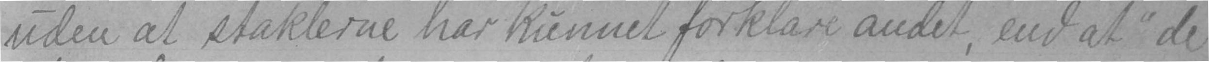uden at staklerne har kunnet forklare andet, end at "de
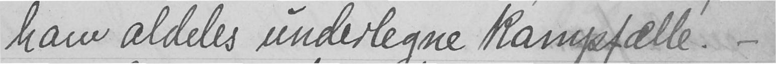ham aldeles underlegne kampfælle. -
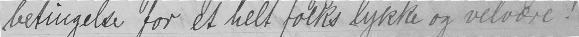betingelse for et helt folks lykke og velvære!
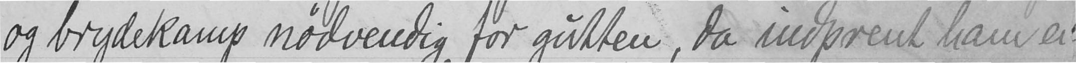og brydekamp nødvendig for gutten, da indprent ham e
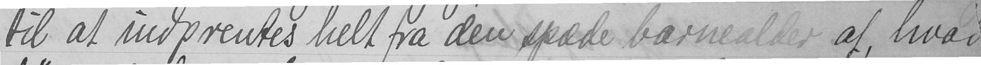til at indprentes helt fra den spæde barnealder af, hva
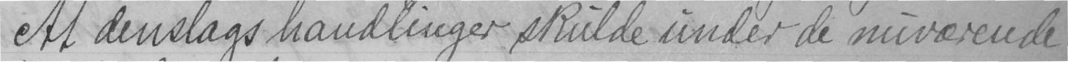At denslags handlinger skulde under de nuværende
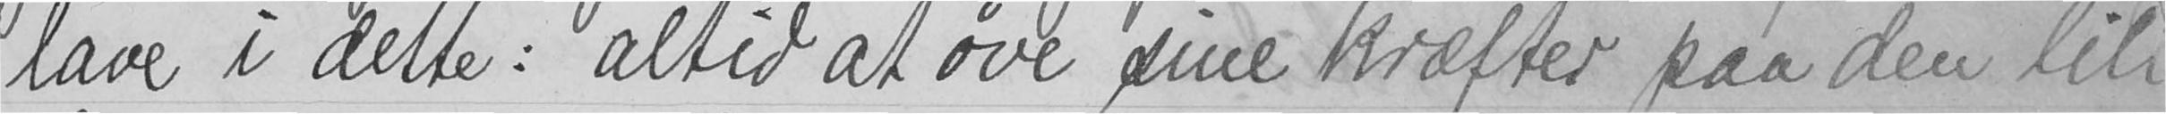lave i dette: altid at øve sine kræfter paa den lil
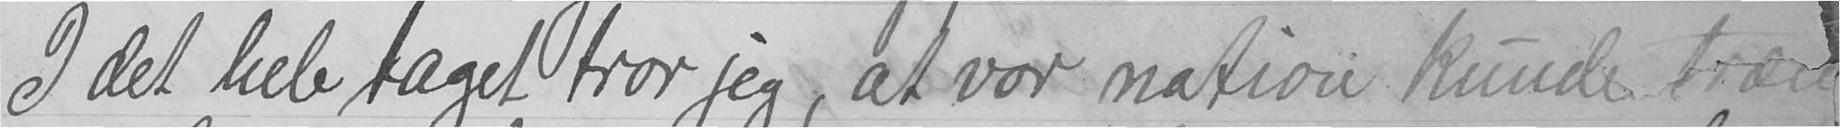I det hele taget tror jeg, at vor nation kunde trænge
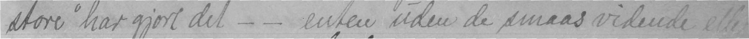store "har gjort det - - enten uden de smaas vidende eller
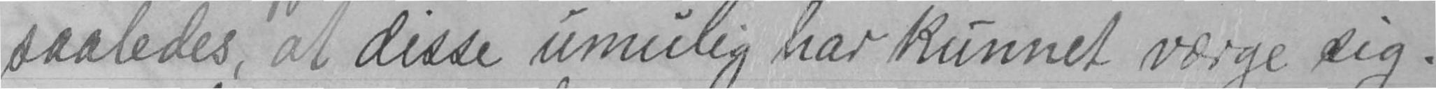saaledes, at disse umulig har kunnet værge sig.
